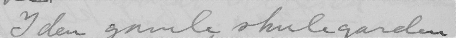I den gamle skulegarden
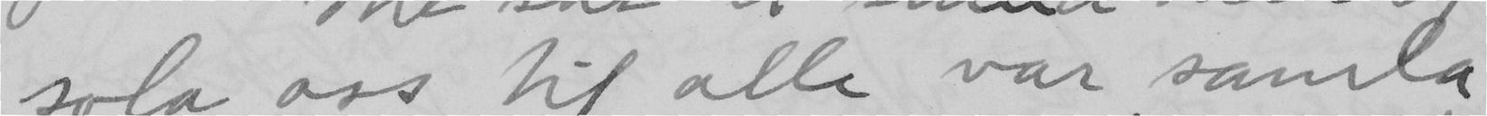sola oss til alle var samla
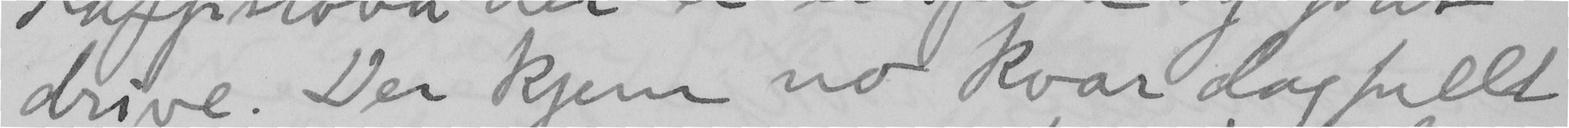drive. Der kjem no kvar dag fullt
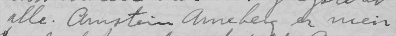alle. Arnstein Arneberg er meir
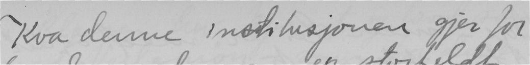Kva denne institusjonen gjer for
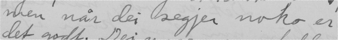men når dei segjer noko er
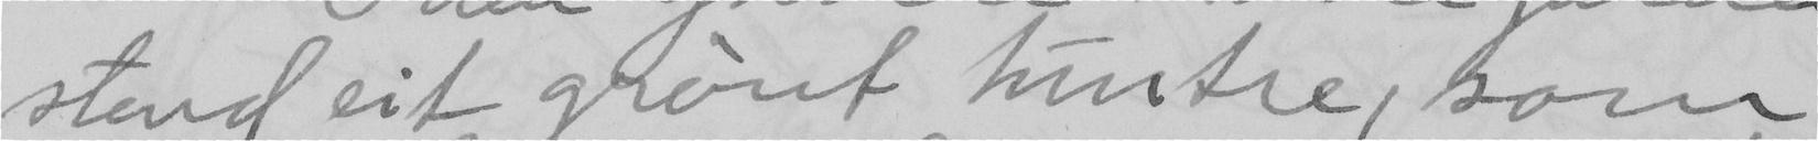stend eit grönt huntre, som
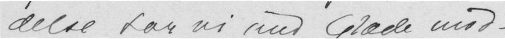delse har vi med Glæde mod-
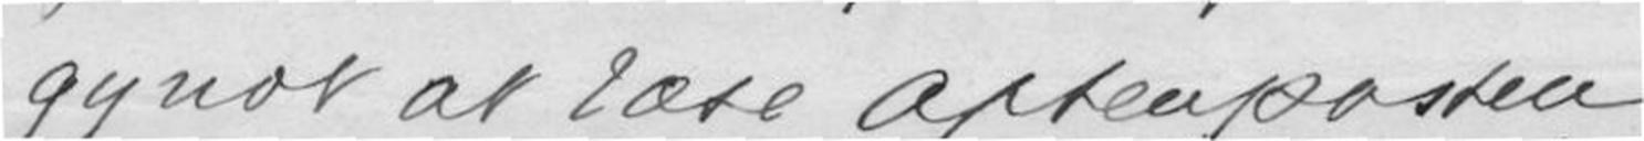gynet at læse Aftenposten
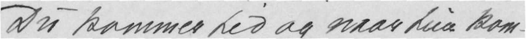Du kommer hid og naar kom-
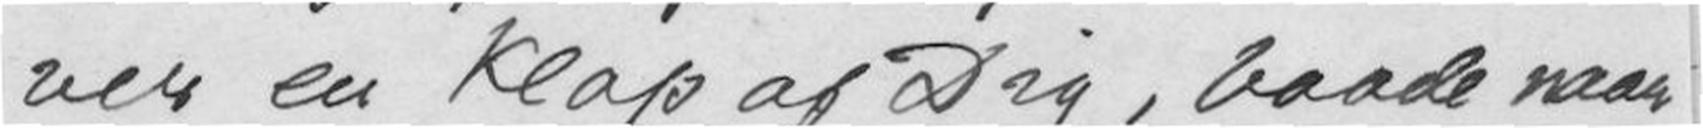ver en Klap af Dig, baade naar
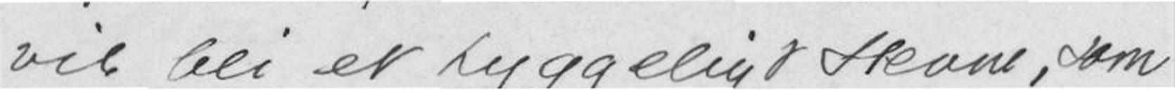vil bli et hyggeligt stevne, som
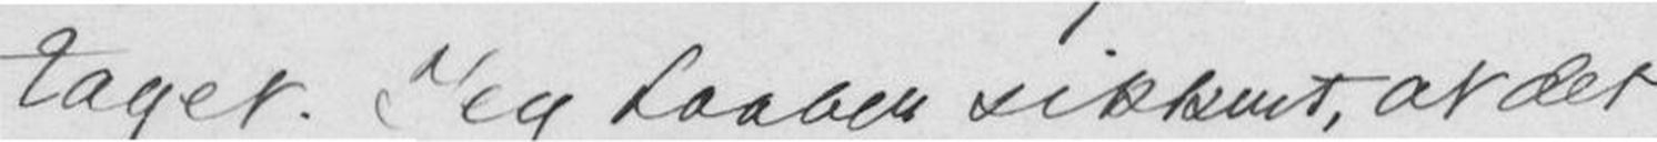taget. Jeg haaber sikkert, at det
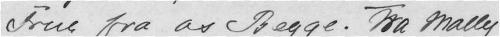Frue fra os Begge. Fra molly
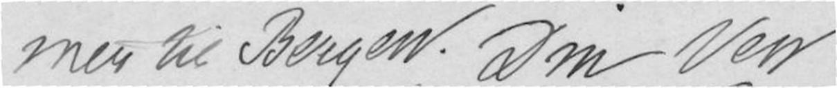mer til Bergen. Din Ven
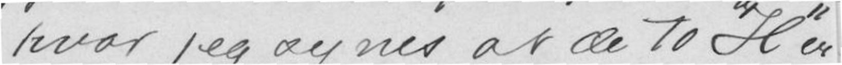hvor jeg synes at de to "H"er
